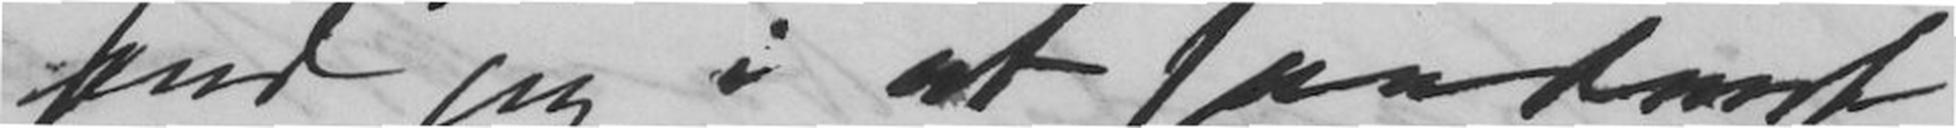end jeg i at saadant
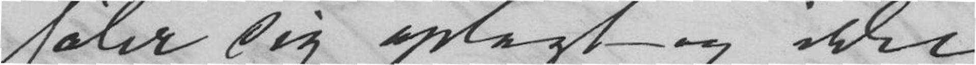føler sig oplagt og ikke
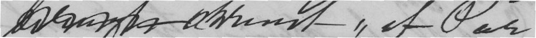skrevet "et Par
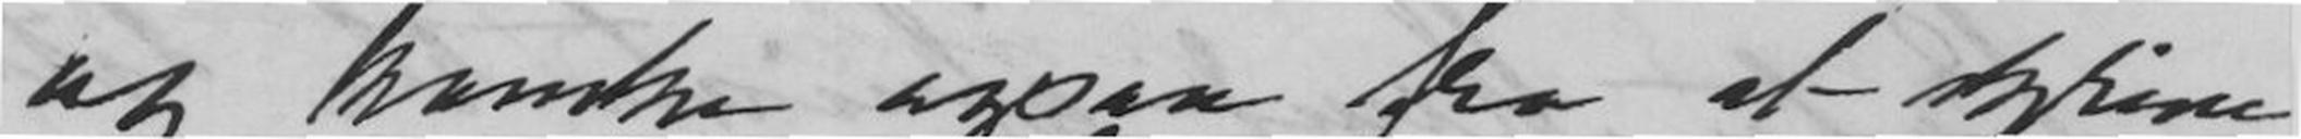og kanske ogsaa fra at skrive
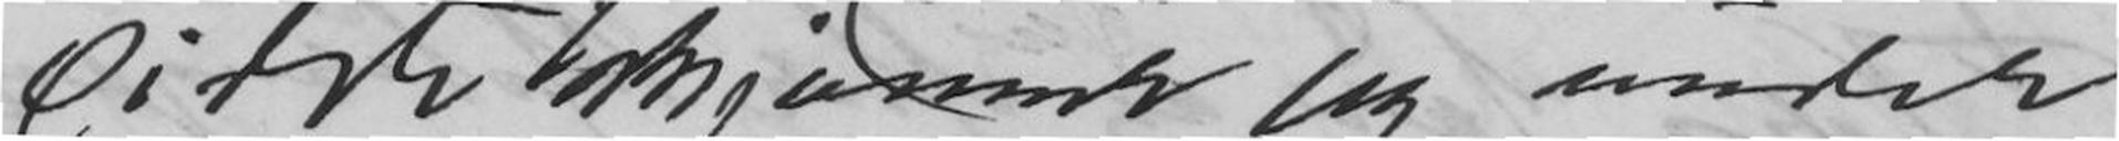sidste skjønner jeg under
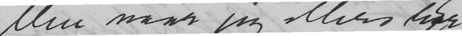Men naar jeg ellers har
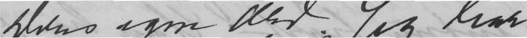Deres egne Ord. Jeg har
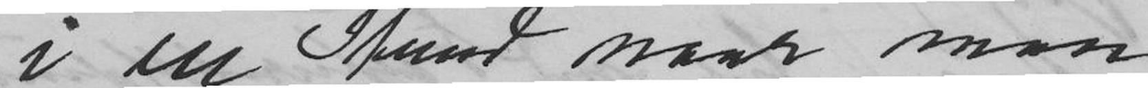i en Stund naar man
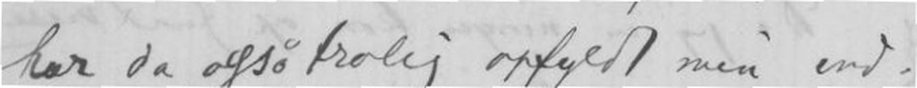har da også trolig opfyldt min ind-
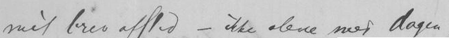mit brev afsted - ikke alene med Dagen,
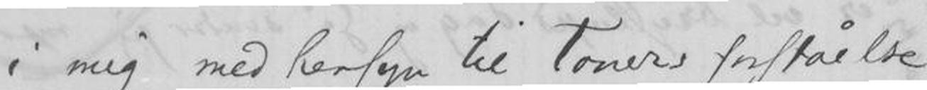i mig med hensyn til toner forståelse
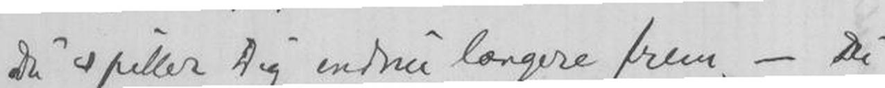Du spiller Dig endnu længere frem, - Du
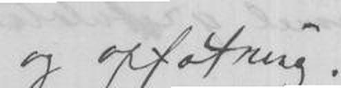og opfatning.
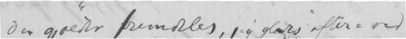Der gjælder fremdeles, jeg glædes oftere ved
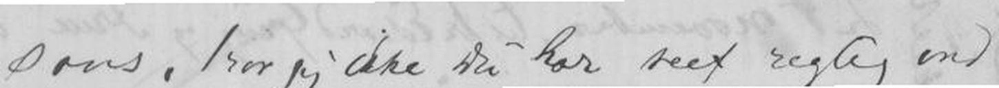sans, tror jeg ikke Du har seet rigtig ind
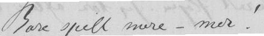Bare spill mere - mer!
